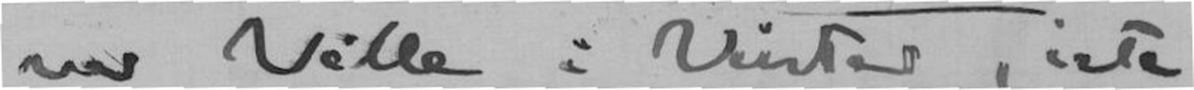vor Villa i Vinter, iste-
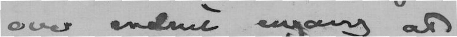over endnu engang at
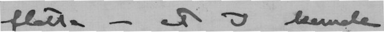flotte - at I kunde
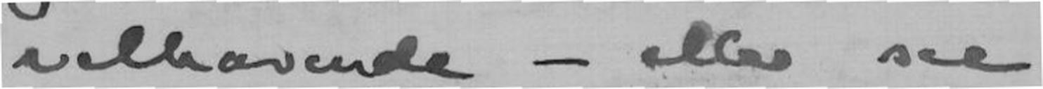velhavende - eller saa
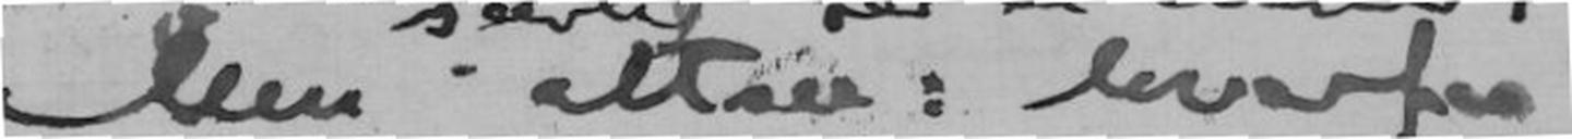Men altsaa: hvorfor
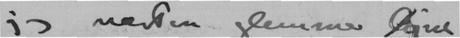jeg næsten glemmer Øjne-
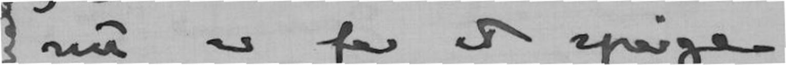nu er for at spørge
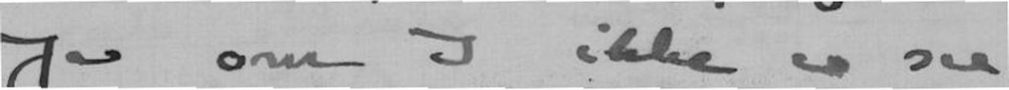Ja om I ikke er saa
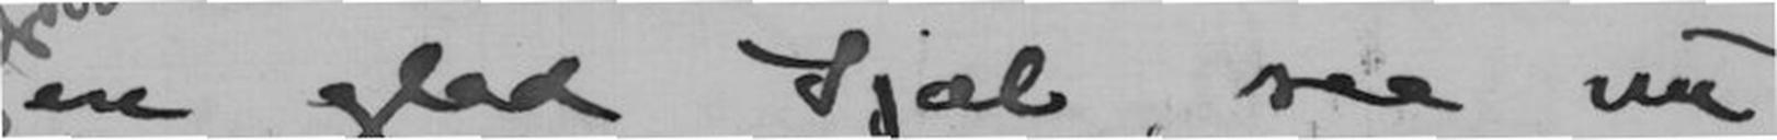en glad Sjæl, saa nu
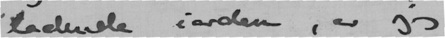ladende iorden, er jeg
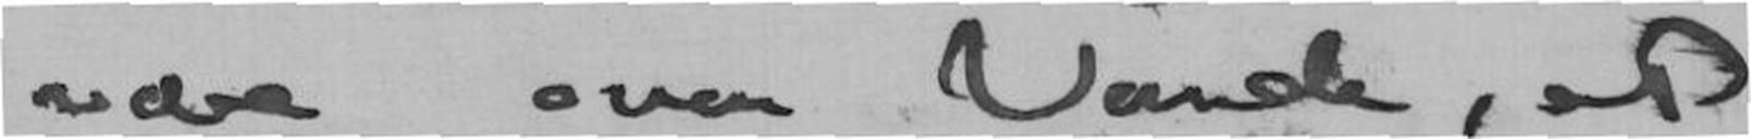være over Vande, at
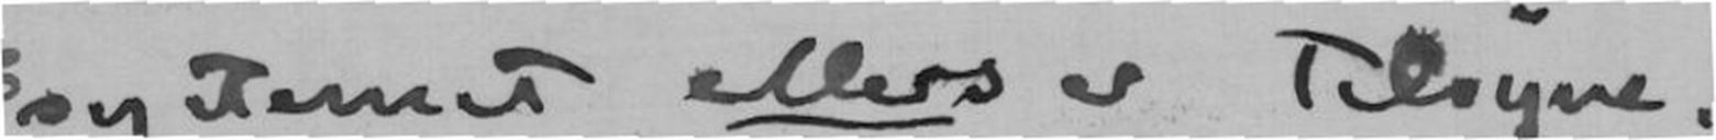systemet ellers er Tilsyne-
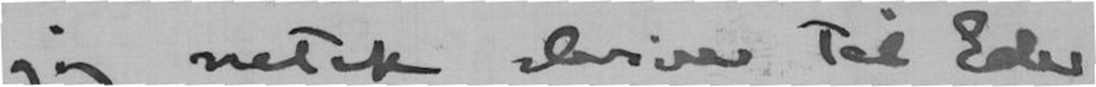jeg netop skriver til Eder
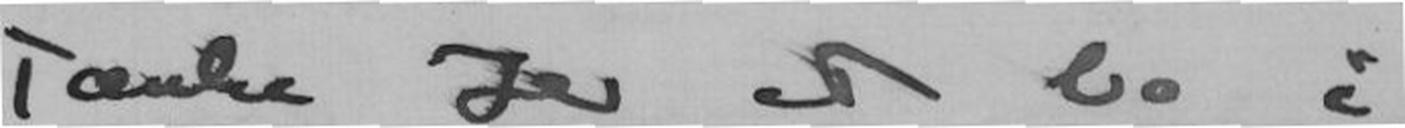tænke Ja at bo i
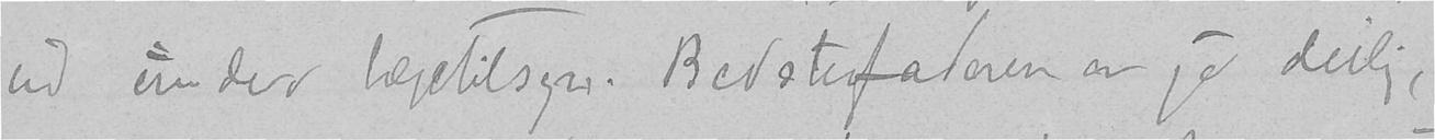ud under lægetilsyn. Bedstefaderen er jo deilig,
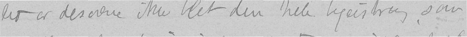det er desværre ikke blet den hele begeistring som
